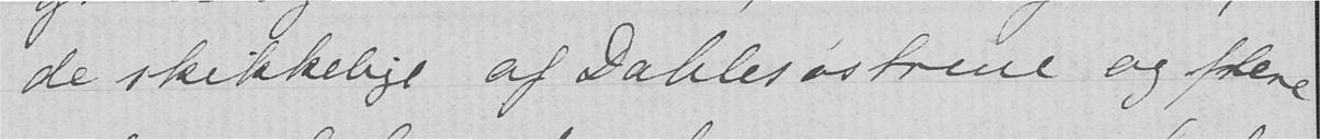de skikkelige af Dahlesøstrene og flere
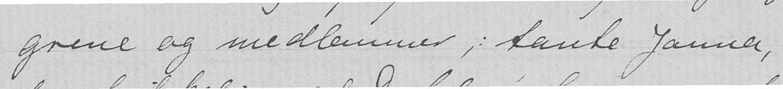grene og medlemmer; tante Janna,
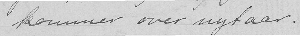kommer over nytaar.
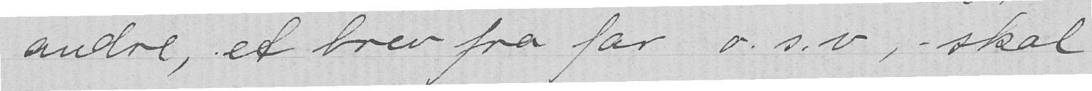andre, et brev fra far o.s.v, - skal
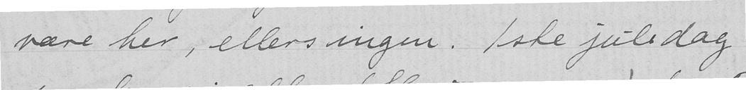være her, ellers ingen. 1ste juledag
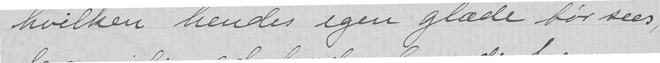hvilken hendes egen glæde bør sees,
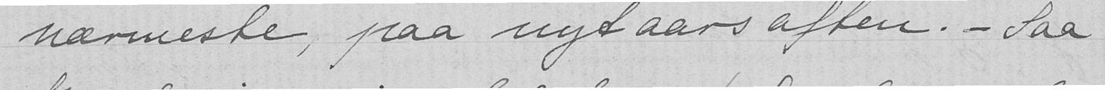nærmeste, paa nytaarsaften. - Saa
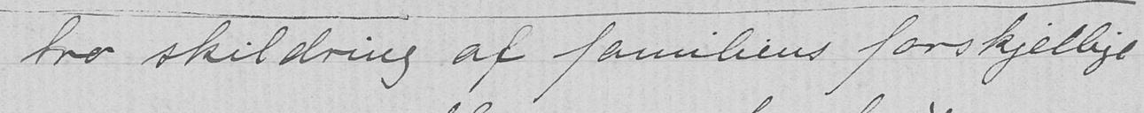tro skildring af familiens forskjellige
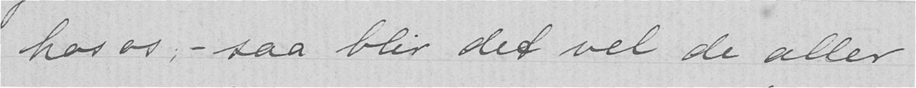hos os, - saa blir det vel de aller
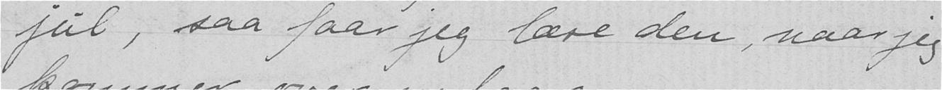jul, saa faar jeg læse den, naar jeg
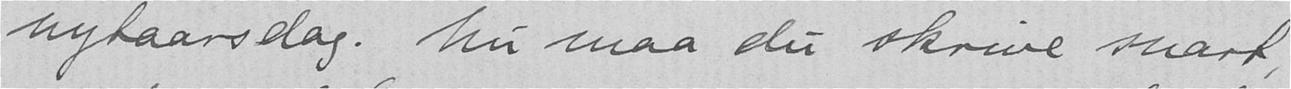nytaarsdag. Nu maa du skrive snart,
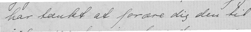har tænkt at forære dig den til
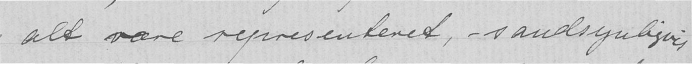alt være representeret, - sandsynligvis
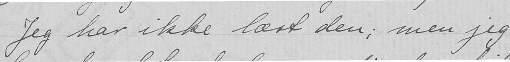Jeg har ikke læst den; men jeg
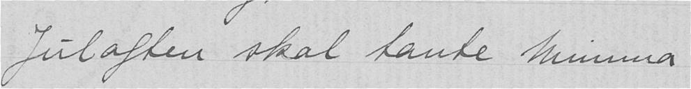- - Julaften skal tante Mimma
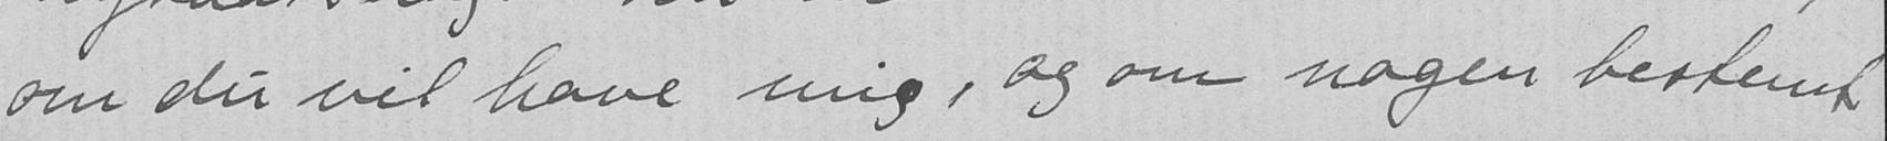om du vil have mig, og om nogen bestemt
